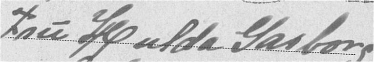Fru Hulde Garborg
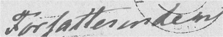Forfatterinden
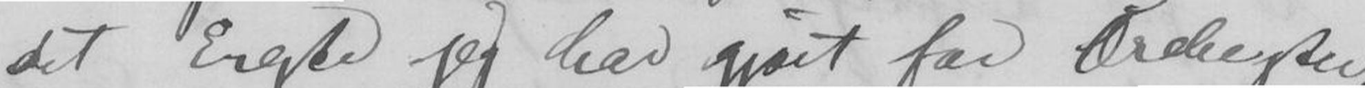det Eneste jeg har gjort for Orchester,
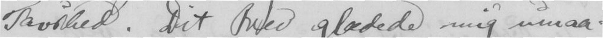Taushed. Dit Brev glædede mig umaa-
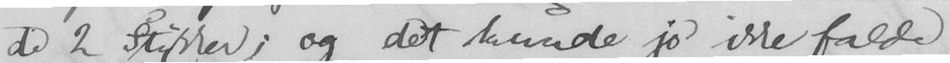de 2 Stykker, og det kunde jo ikke falde
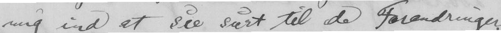mig ind at see surt til de Forandringer,
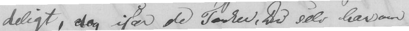deligt, dog især de Tanker, Du selv har om
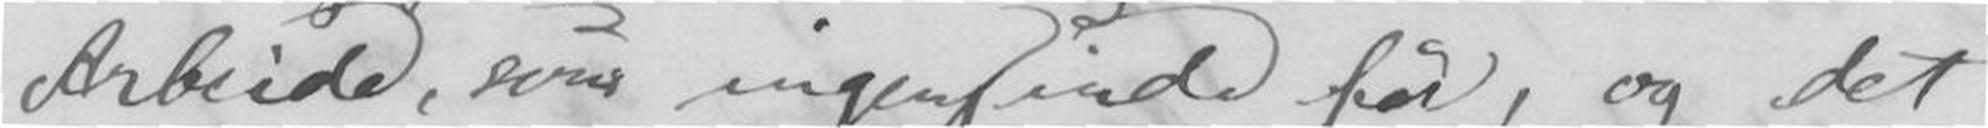Arbeide, som ingensinde før, og det
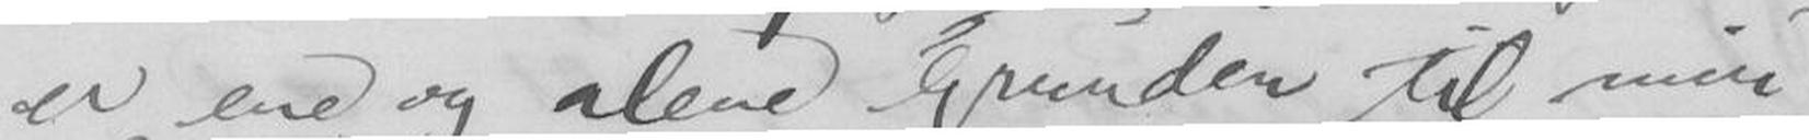er ene og alene Grunden til min
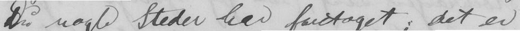Du nogle Steder har foretaget, det er
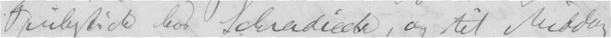Frühstück hos Schradieck, og til Middag
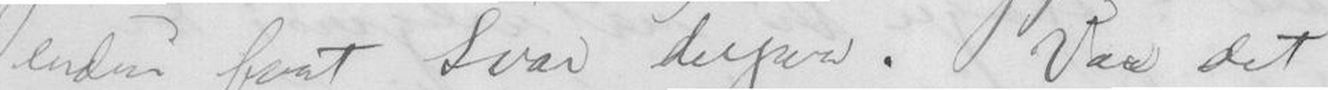endnu faat Svar derpaa. Var det
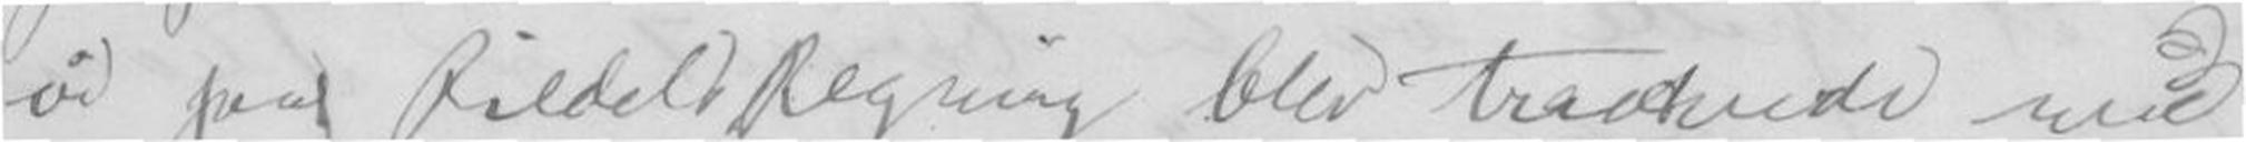vi paa Riedels Regning blev traterede med
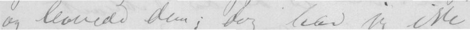og leverede dem; dog har jeg ikke
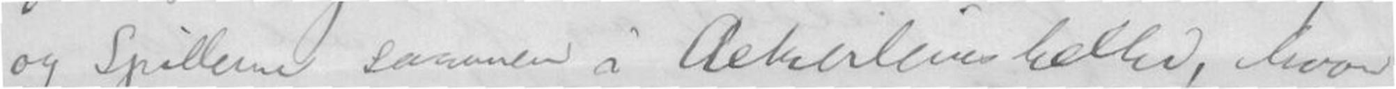og Spillerne sammen i Ackerleinskeller hvor
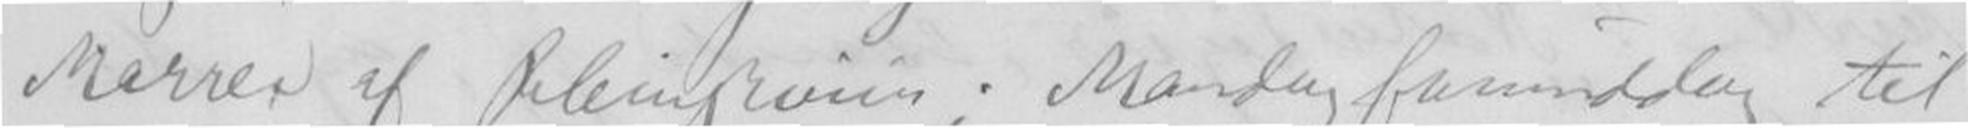Masser af Rihnskvin; Mandag formiddag til
